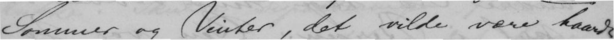Sommer og Vinter, det vilde være haardt
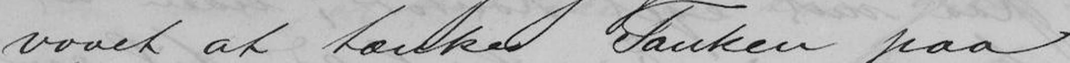vovet at tænke Tanken paa
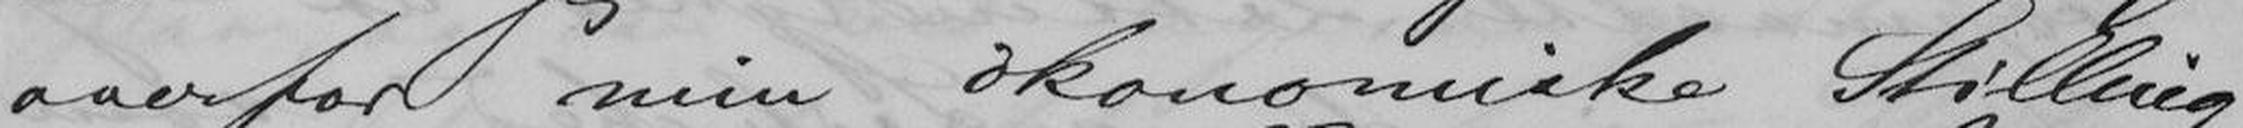overfor min økonomiske Stilling
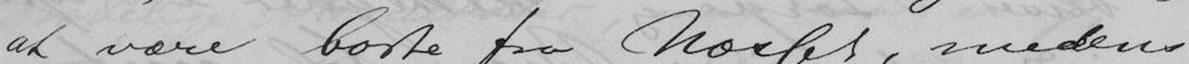at være borte fra Næsset, medens
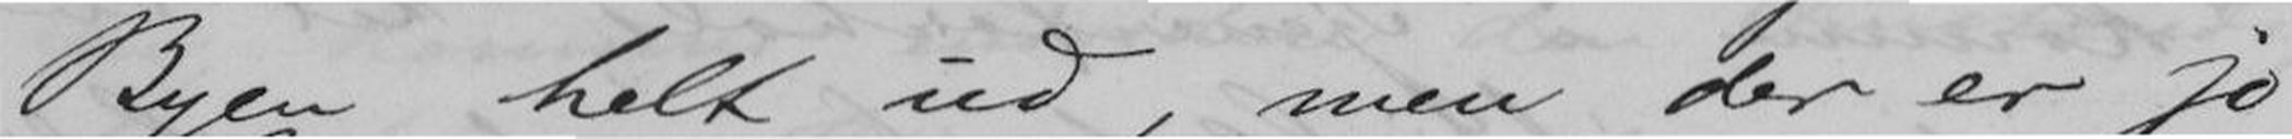Byen helt ud, men der er jo
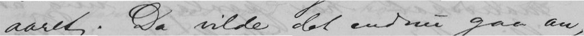aaret. Da vilde det endnu gaa an,
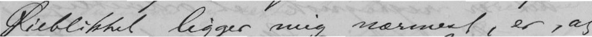Øieblikket ligger mig nærmest, er, at
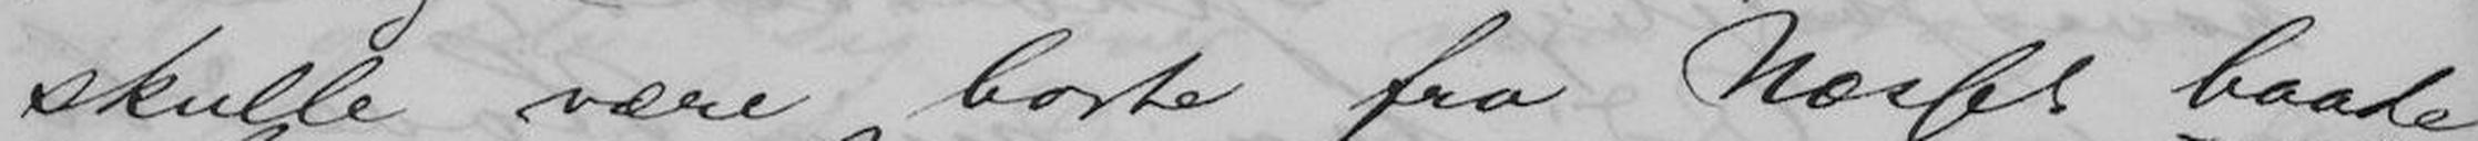skulle være borte fra Næsset baade
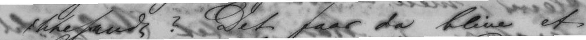ikke sandt? Det faar da blive et
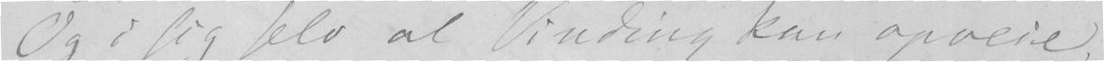Og i sig selv al Vinding kan opveie.
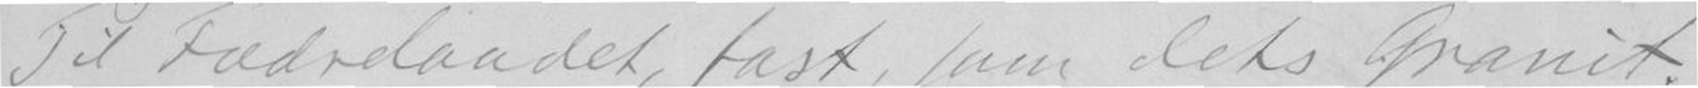Til Fædrelandet, fast, som dets Granit.
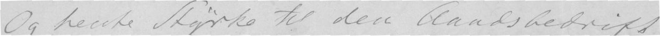Og hente Styrke til den Aandsbedrift
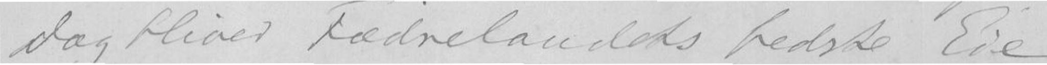Dog bliver Fædrelandets bedste Eie
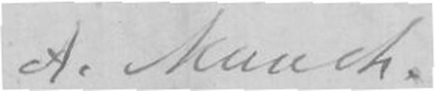A. Munch.
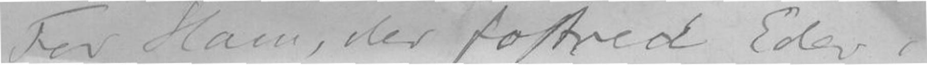For Ham, der fostred Eder,
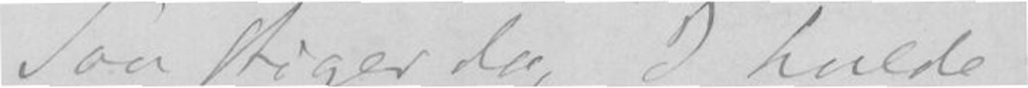Saa stiger da, I hulde
